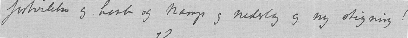fortvilelse og haab og kamp og nederlag og ny stigning!
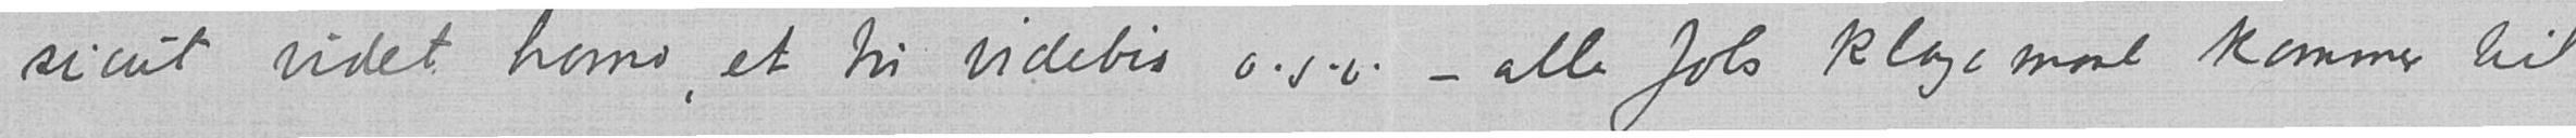sicut videt homo, et tu videbis o.s.v. – alle Jobs klagemaal kommer til
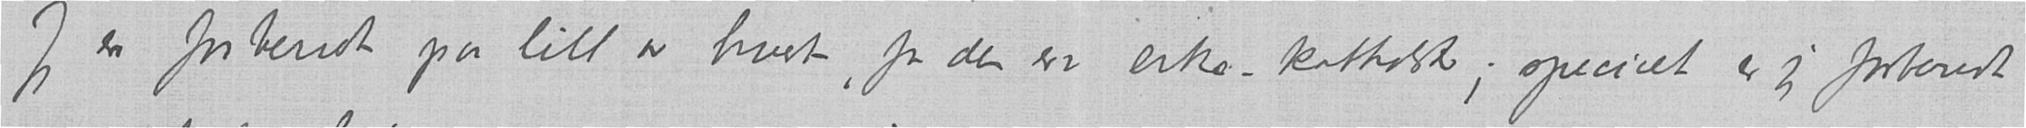Jeg er forberedt paa litt av hvert, for den er erke-katholsk; specielt er jeg forberedt
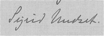Sigrid Undset.
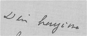Din hengivne
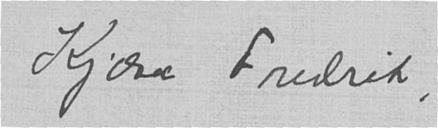Kjære Fredrik,
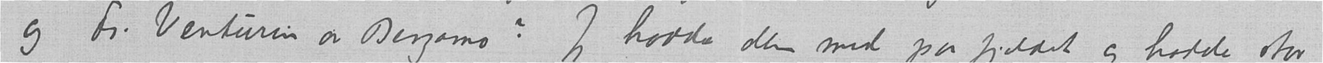og Fr. Venturin av Bergamo? Jeg hadde dem med paa fjeldet og hadde stor
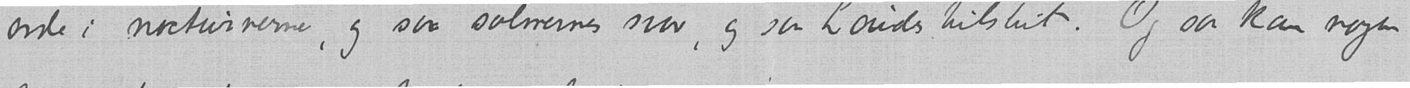orde i nocturnerne, og saa salmernes svar, og saa Lauds tilslut. Og saa kan nogen
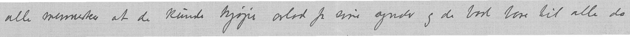alle mennesker at de kunde kjøpe avlad for sine synder og de bad bare til alle de
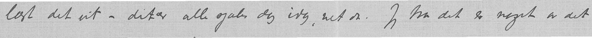læst det ut – det er alle sjæles dag idag, vet du. Jeg tror det er noget av det
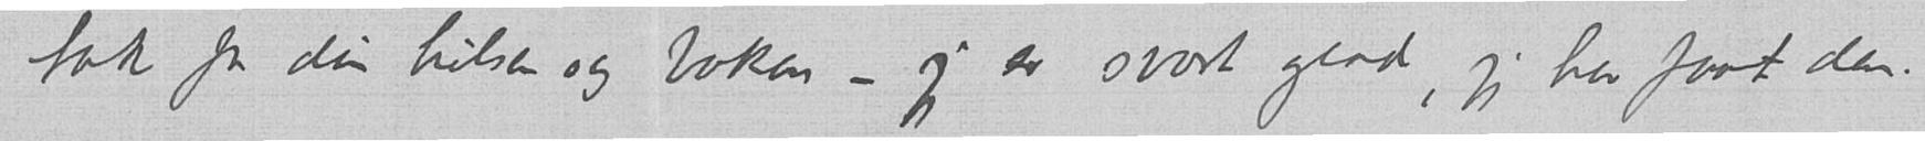tak for din hilsen og boken – jeg er svært glad, jeg har faat den.
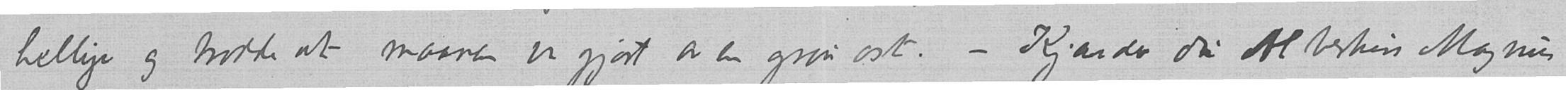hellige og trodde at maanen var gjort av en grøn ost. - Kjænder du Albertus Magnus
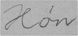Høn
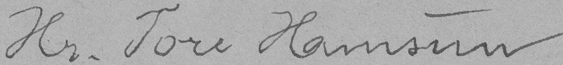Hr. Tore Hamsun
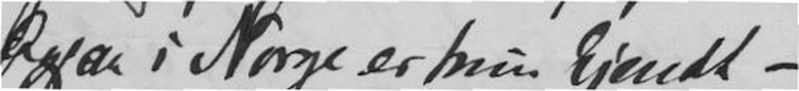Ogsaa i Norge er hun kjendt - -
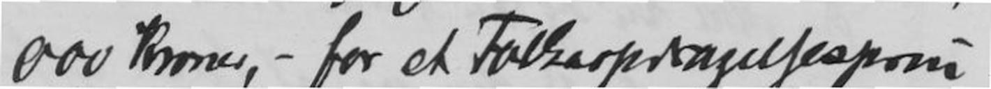000 kroner, - for et Folkeopdragelseprin-
